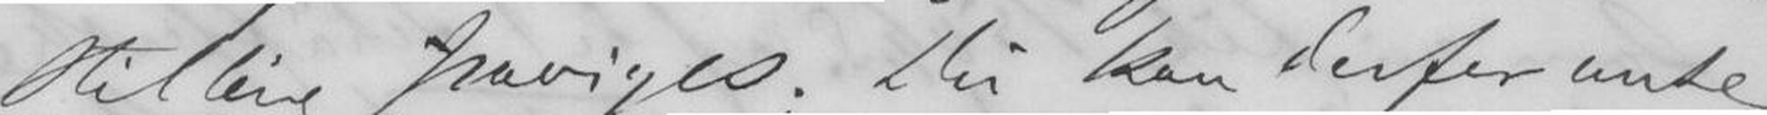stilling fraviges; Du kan derfor vente
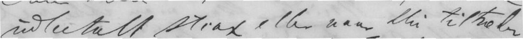udbetalt strax eller naar Du tiltræder
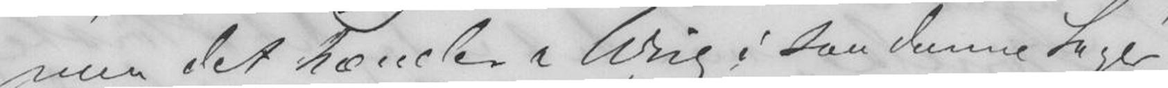men det hænder aldrig i saadanne Sager
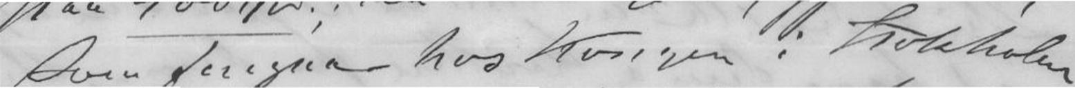som foregaar hos Kongen i Stockholm
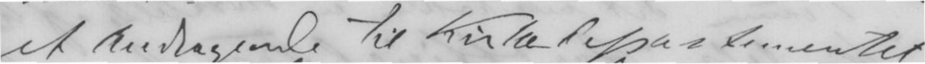et Andragende til Kirkedepartementet
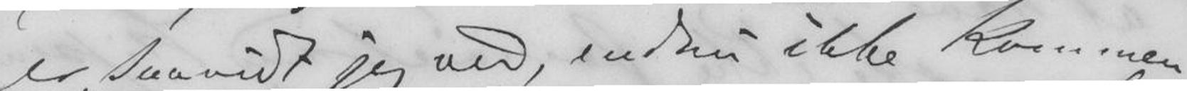er saavidt jeg ved, endnu ikke kommen
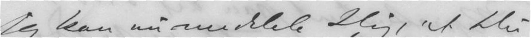Jeg kan nu meddele Dig, at Du
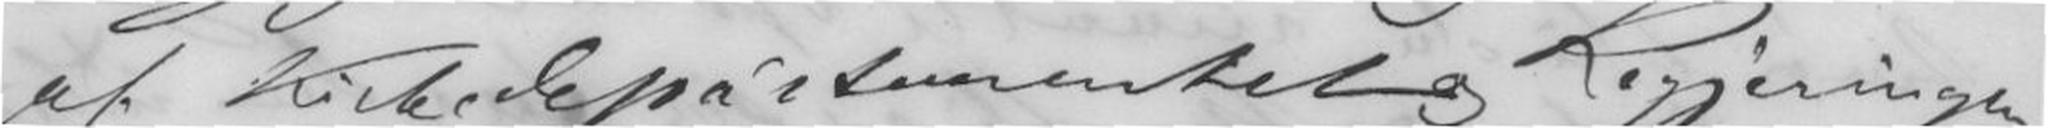af Kirkedepartementet og Regjeringen
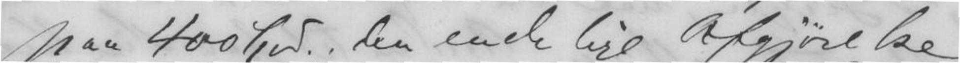paa 400Spd.; den endelige Afgjørelse
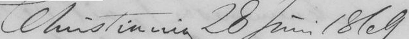Christiania 28 Juni 1869.
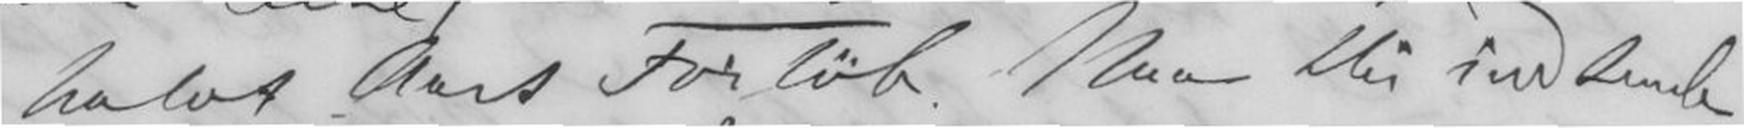halvt Aars Forløb. Naar Du indsende
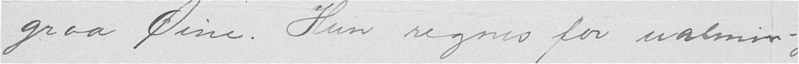graa Øine. Hun regnes for ualmindelig-
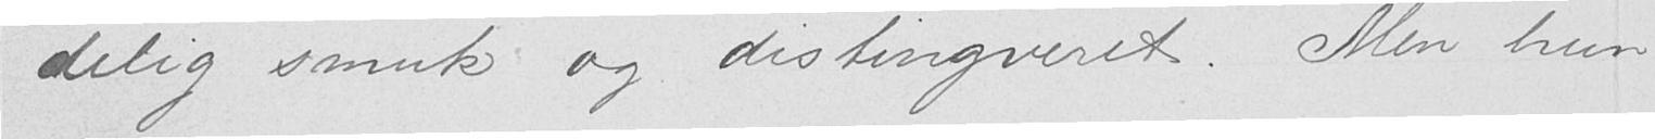delig smuk og distingveret. Men hun
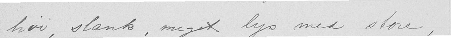høi, slank, meget lys med store,
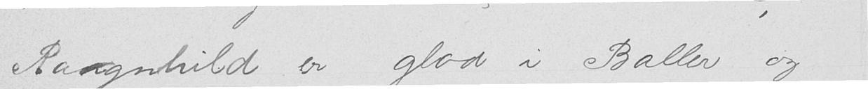Raagnhild er glad i Baller og
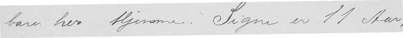bare her Hjemme. Signe er 11 Aar,
